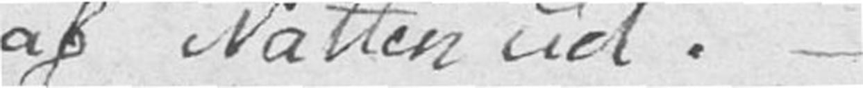af Natten ud. –
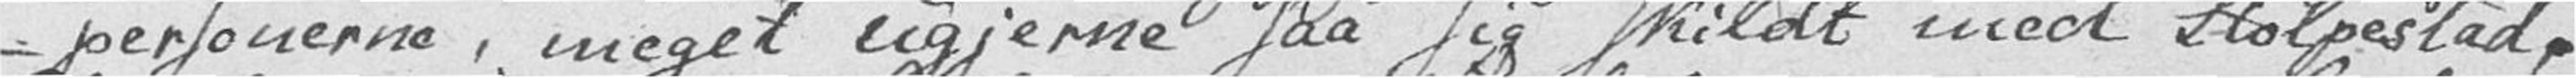-personerne, meget ugjerne saa sig skildt med Stolpestad.
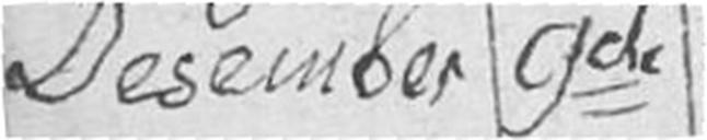Desember 9de
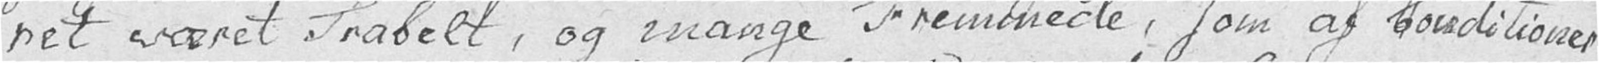ret været Trabelt, og mange Fremmede, som af Condisjoner
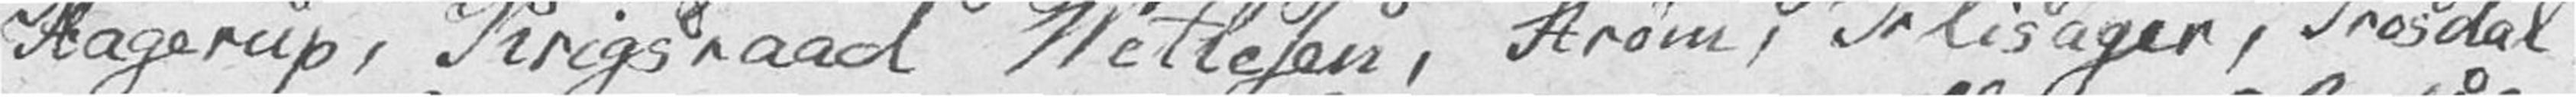Hagerup, Krigsraad Vetlesen, Strøm, Flisager, Trosdal
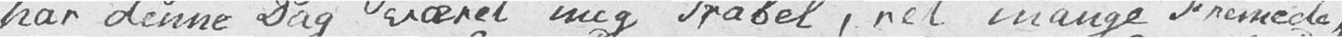har denne Dag været mig Trabel, ret mange Fremmede,
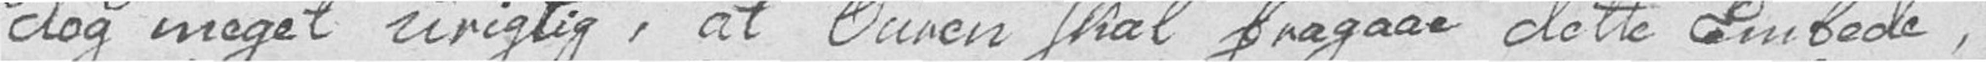dog meget urigtig, at Ouren skal fragaae dette Embede,
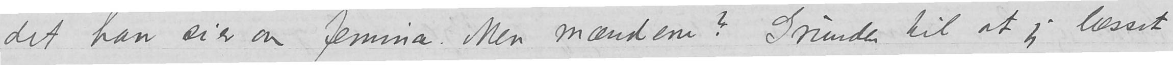det han sier om femina. Men mændene? Grunden til at jeg læsset
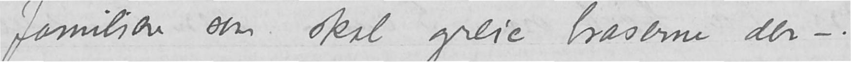familien som skal greie braserne der –.
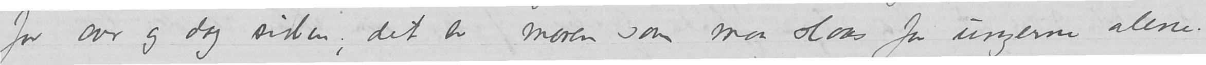for aar og dag siden; det er moren som maa slaas for ungerne alene.
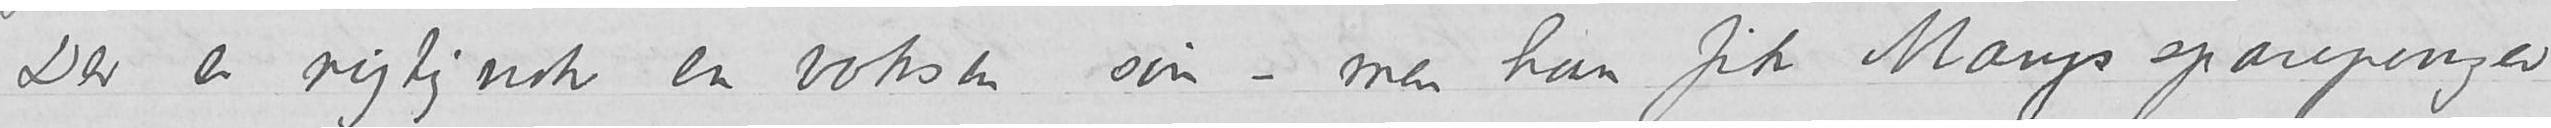Der er rigtgnok en voksen søn – men han fik Marys sparepenger
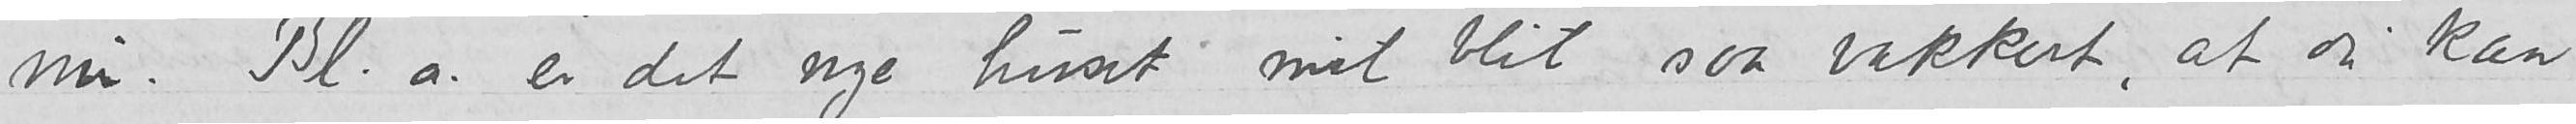nu. Bl.a. er det nye huset mit blit saa vakkert, at du kan
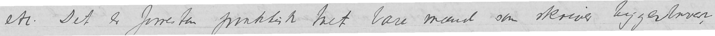etc. Det er forresten praktisk talt bare mænd som skriver tiggerbrever,
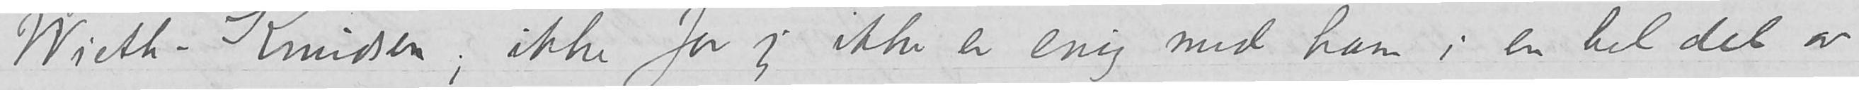Wieth-Knudsen; ikke for jeg ikke er enig med ham i en hel del av
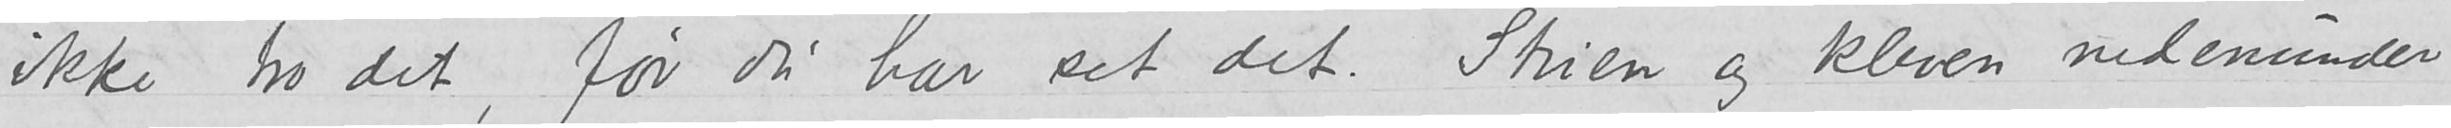ikke tro det, før du har set det. Stuen og kleven nedenunder
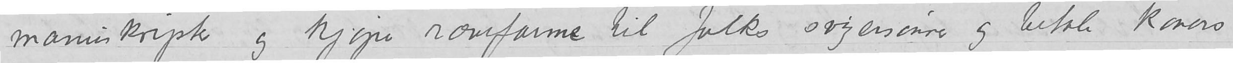manuskripter og kjøpe rævefarmer til folks svigersønner og betale koners
